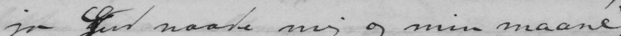jo Gud naade mig og min "Maane",
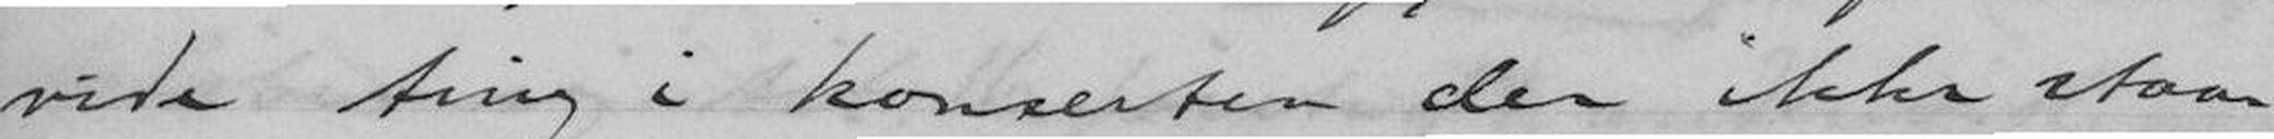vide ting i konserten der ikke staar
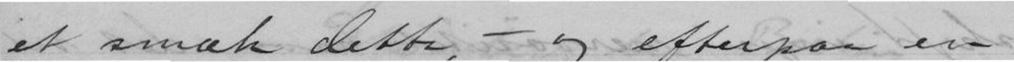et smæk dette, - og etterpaa en
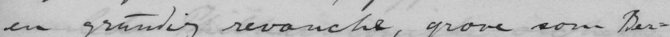en grundig revanche, grove som Ber-
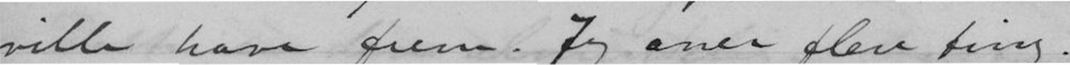ville have frem. Jeg aner flere ting.
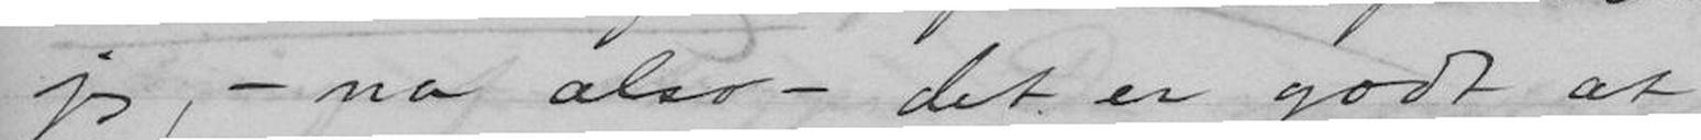jeg, - na also - det er godt at
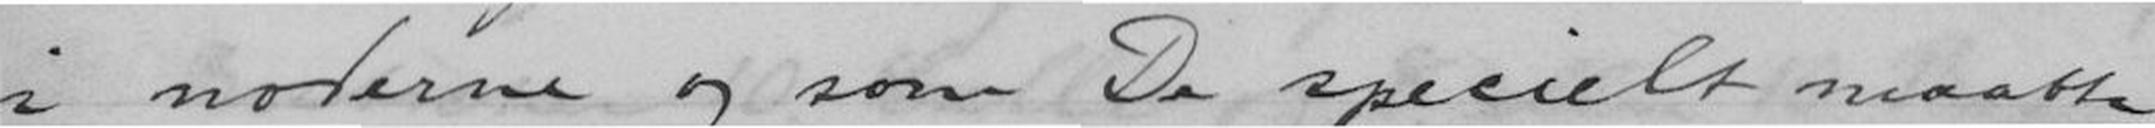i noderne og som De specielt maatte
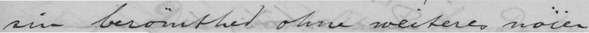sin berømthed ohne weiteres nöier
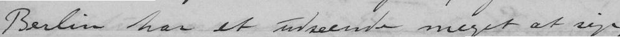Berlin har et udseende meget at sige,
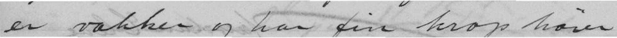er vakker og har fin krop hører
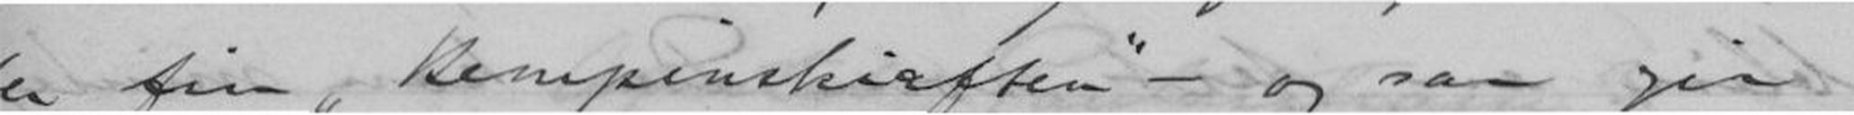fin "Kempinskiaften" - og saa gir
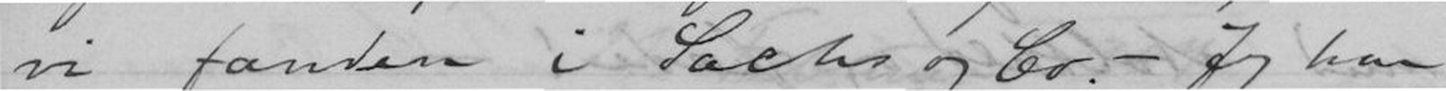vi fanden i Sachs og Co. - Jeg har
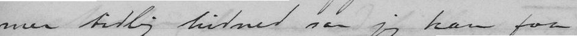mer tidlig hidned saa jeg kan faa
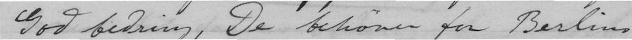God bedring, De behøver for Berlins
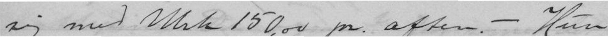sig med Mrk 150,00 pr. aften. - Hun
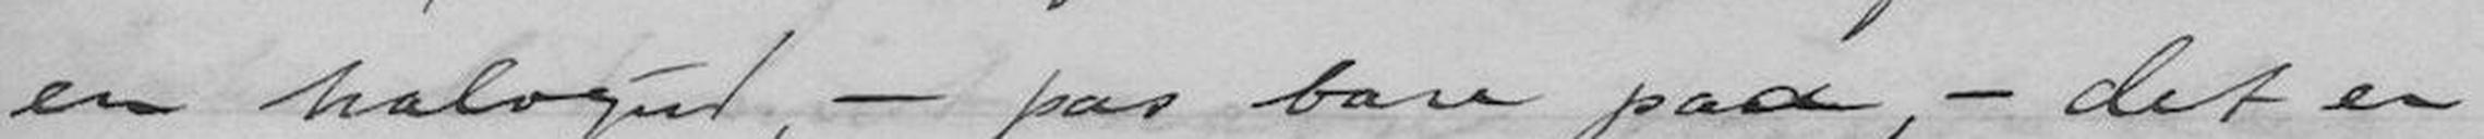en halvgud, - pas bare paa, - det er
